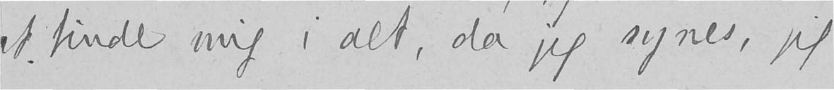affinde mig i alt, da jeg synes, jeg
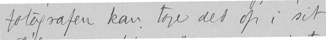fotografen kan tage det op i sit
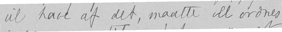vil have af det maatte vel ordnes
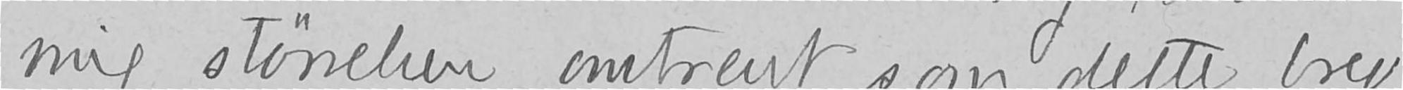mig størrelsen omtrent, som dette brev-
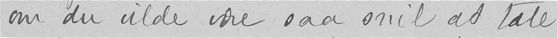om du vilde være saa snil at tale
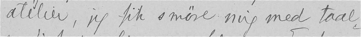atélier, jeg fik smøre mig med taal-
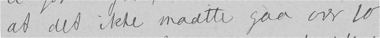at det ikke maatte gaa over 10
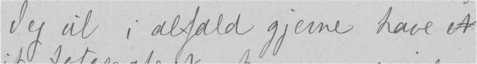Jeg vil i alfald gjerne have et
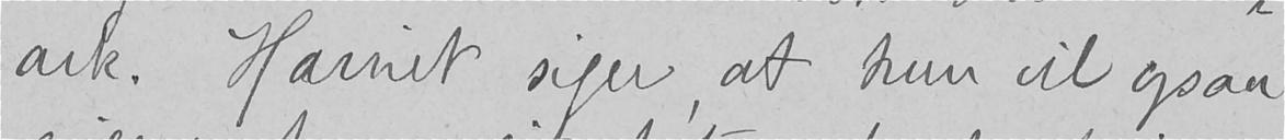ark. Harriet siger, at hun vil ogsaa
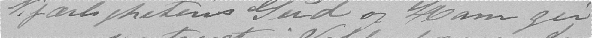kjærlighetens Gud og Ham gir
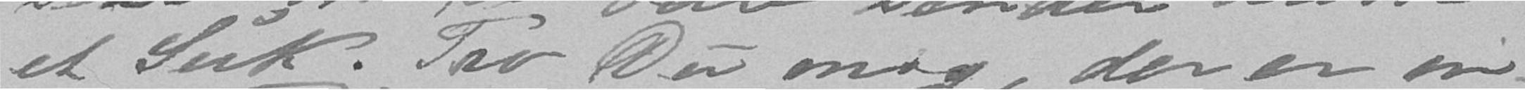et Suk. Tro Du mig, der er in-
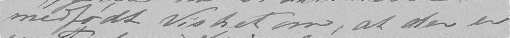medfødt Vishet om, at der er
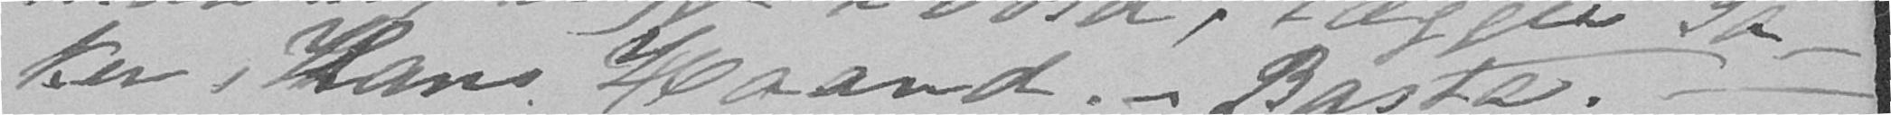ker, Hans Haand. - Baste. -
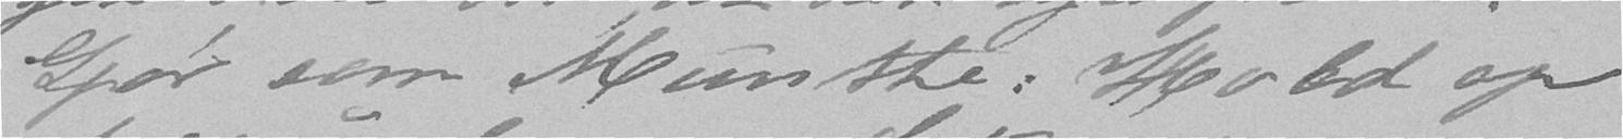Gjør som Munthe: Hold op
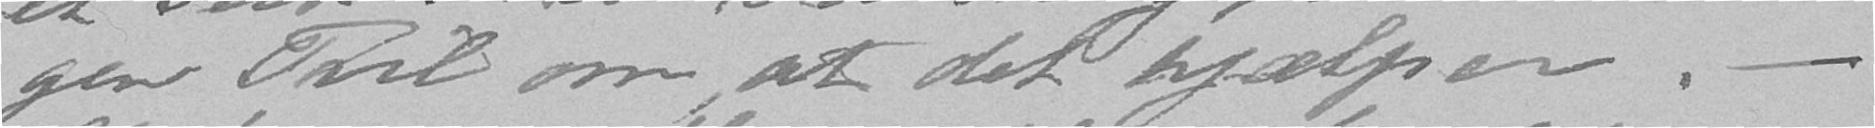gen Tvil om at det hjælper. -
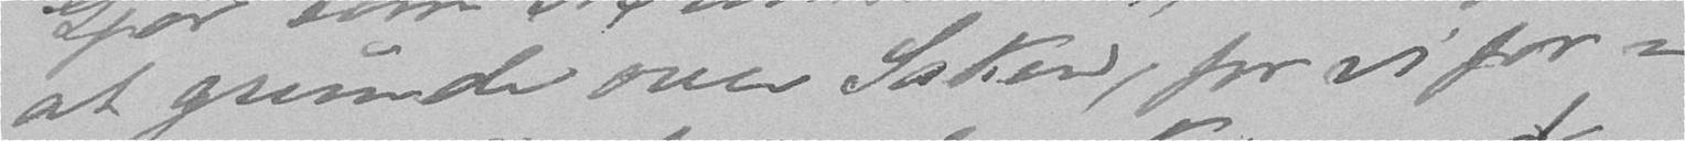at grunde over Saken, for vi for-
